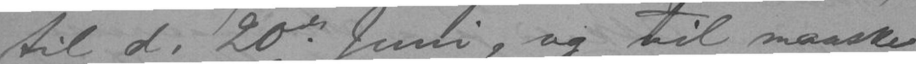til d. 20de Juni, og vil maaske
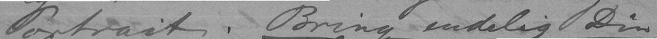Portrait. Bring endelig Din
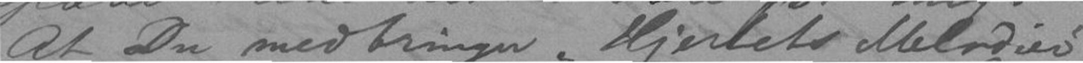At Du medbringer Hjertets Melodier,
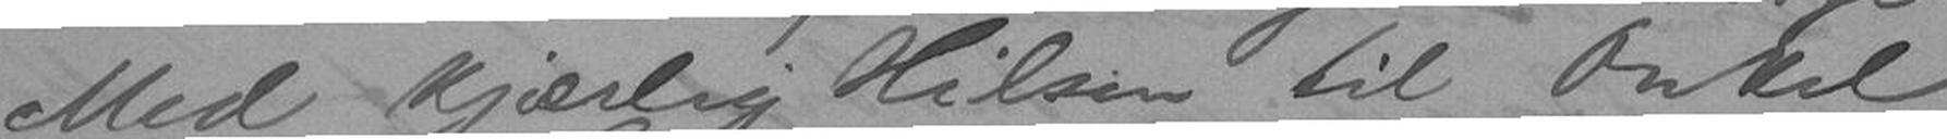Med kjærlig Hilsen til Onkel,
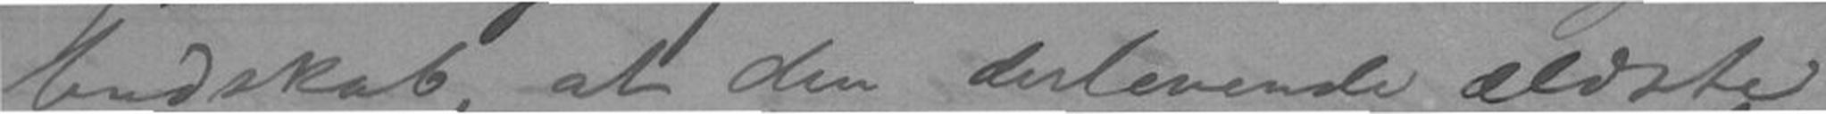budskab, at den derlevende ældste
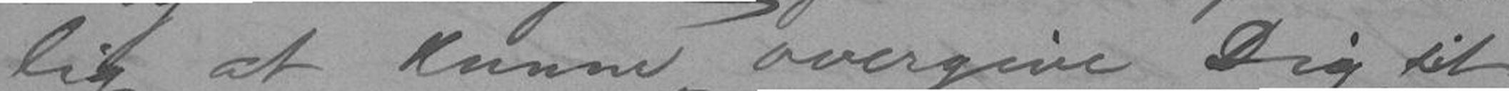ligt at kunne overgive Dig sit
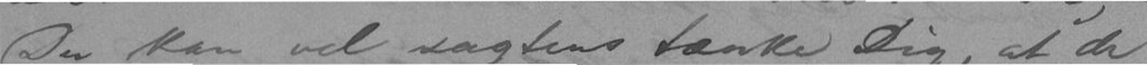Du kan vel sagtens tænke Dig, at de
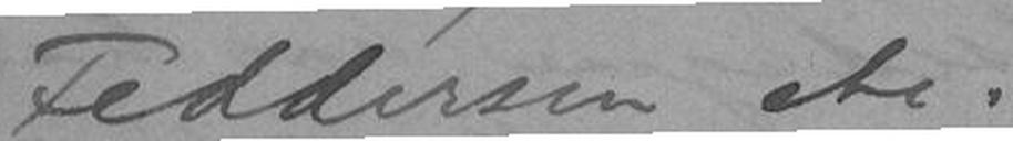Feddersen etc.
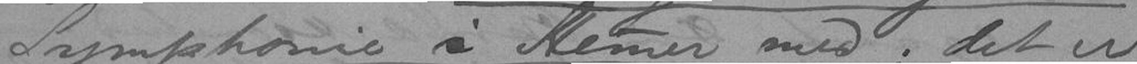Symphonie i Stemmer med; det er
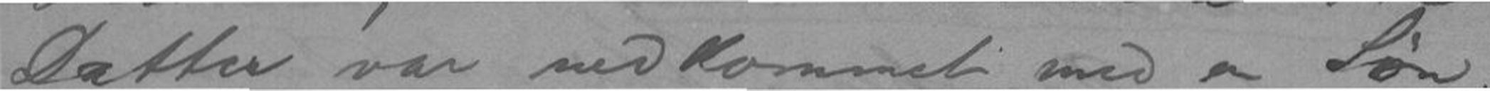Datter var nedkommet med en Søn, -
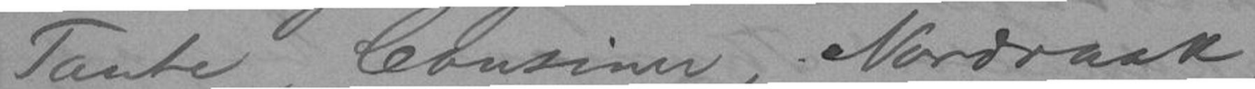Tante, Cousiner, Nordraak,
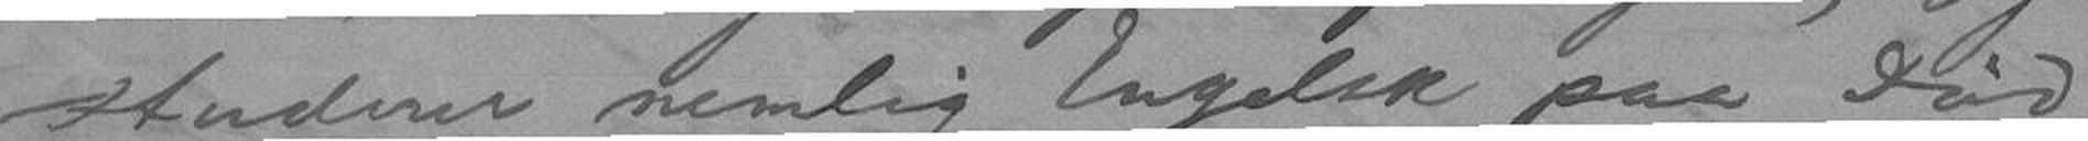studerer nemlig Engelsk paa Død
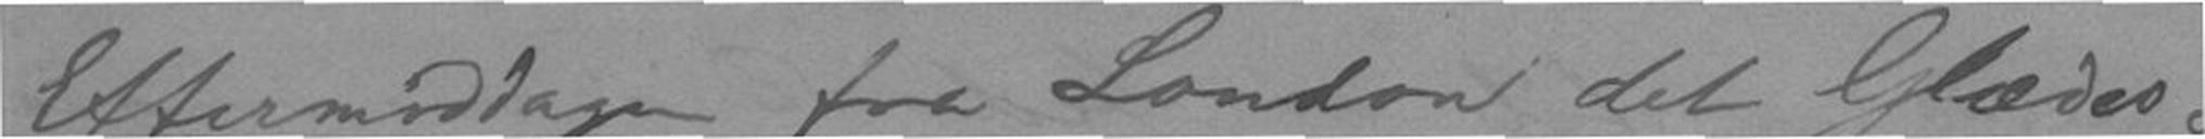Eftermiddagen fra London det Glædes-
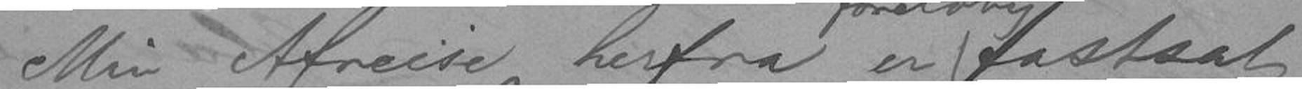Min Afreise herfra er fastsat
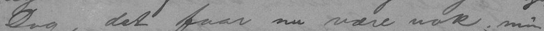Dog, det faar nu være nok; min
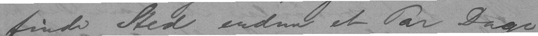finde Sted endnu et Par Dage
